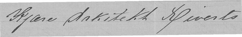Kjære Arkitekt Riverts
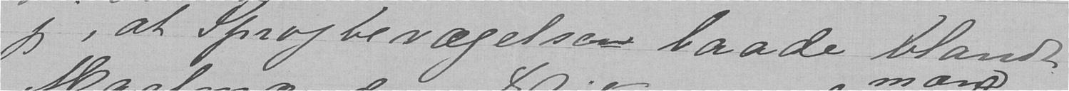jeg, at Sprogbevægelsen baade blandt
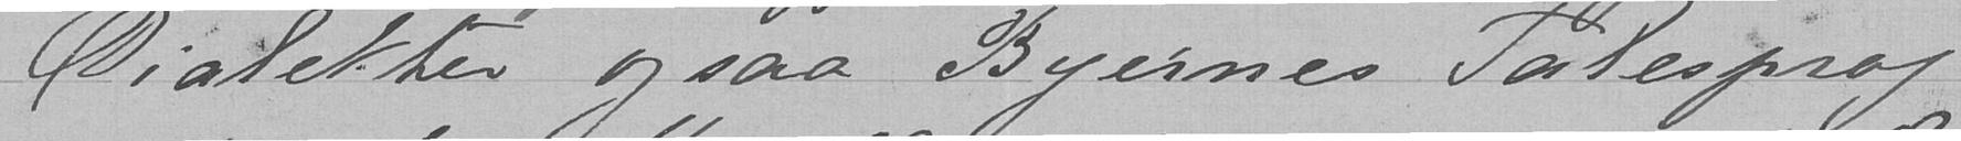Dialekter, ogsaa Byernes Talesprog
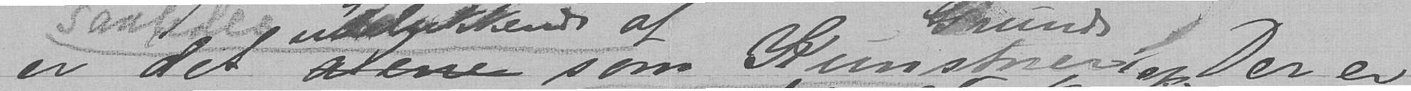er det alene som Kunstneriske Der er
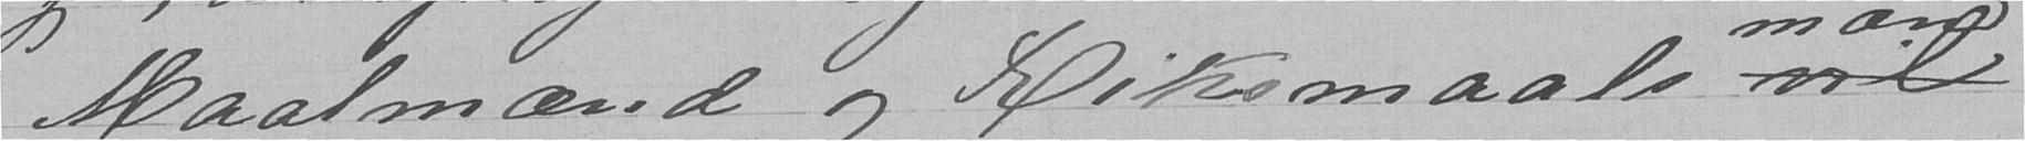Maalmænd og Ritesmaals vil
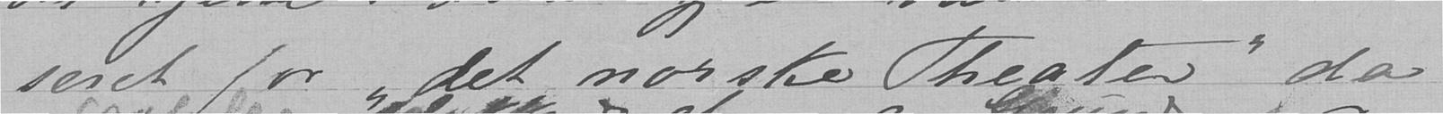seret for det norske Theater da
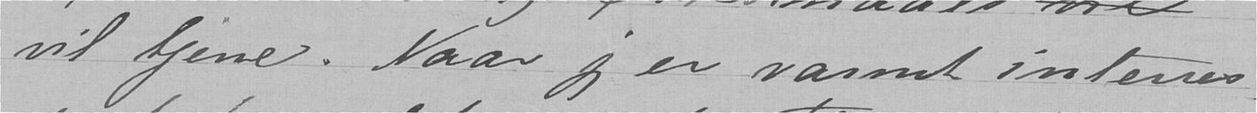vil tjene. Naar jeg er varmt interres-
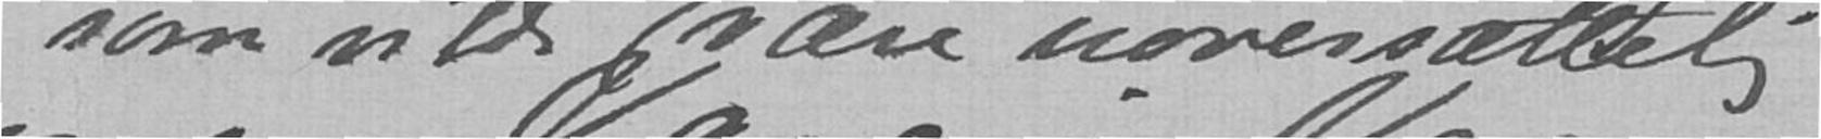som vilde være uoversættelig
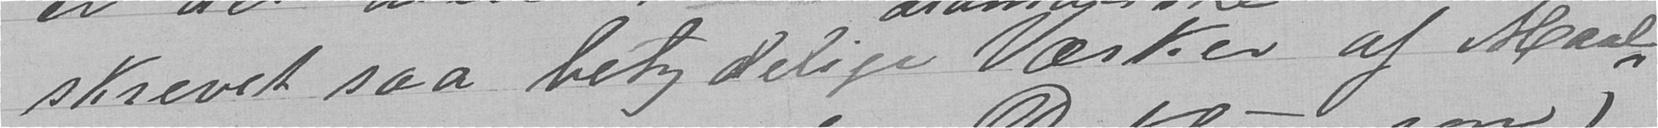skrevet saa betydelige Værker af Maal-
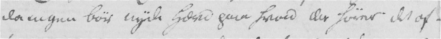da ingen bør nyde Hævd paa hvad der hører det of-
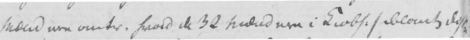Mænd ere omtr. hvad de 32 Mænd ere i Kiøbh :/ iblandt disse
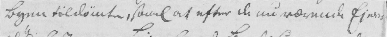Byen tildømte, saal at efter de nu værende Eieres
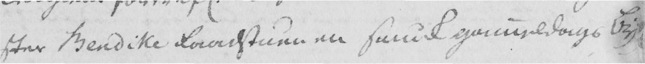ster Bendike Raadstuen en smuck gameldags Byg-
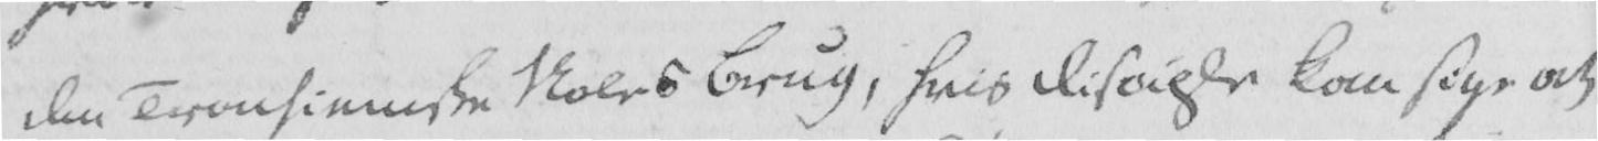den Tronhiemske Skoles Brug, hvis Disiple kan sige at
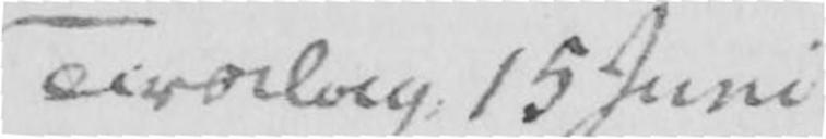Tirsdag, 15 Juni
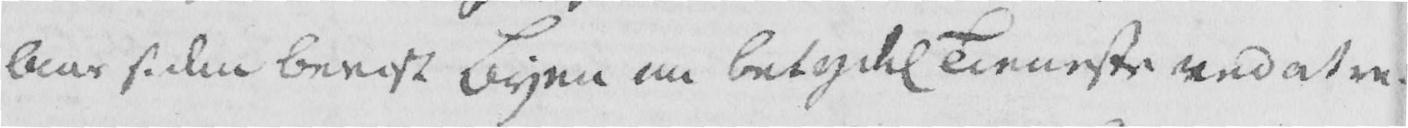Aar siden bevist Byen en betydel Tieneste ved at re-
